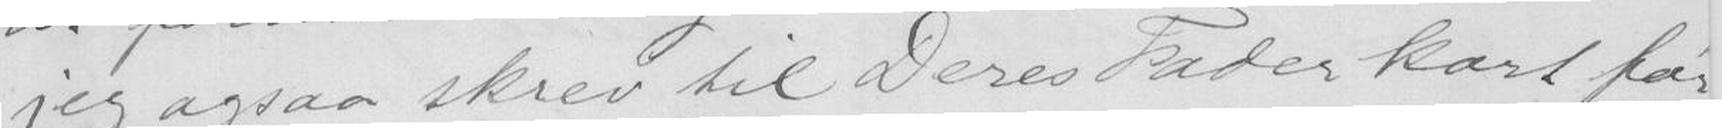jeg ogsaa skrev til Deres Fader kort
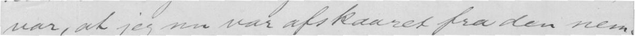var, at jeg nu var afskaaret fra den nem-
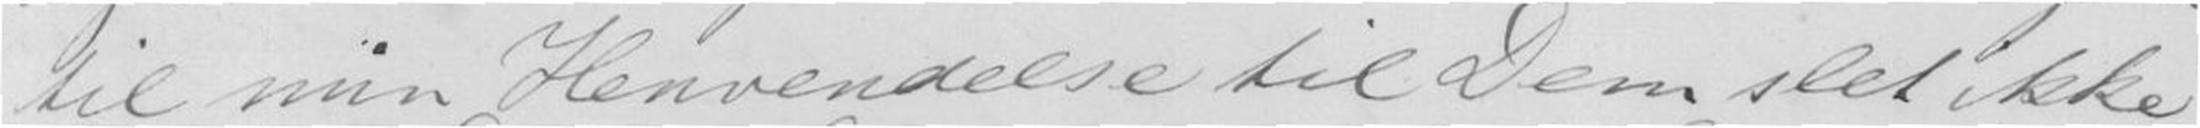til min Henvendelse til Dem slet ikke
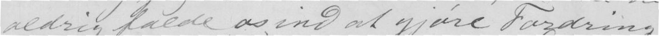aldrig falde os ind at gjøre Fordring
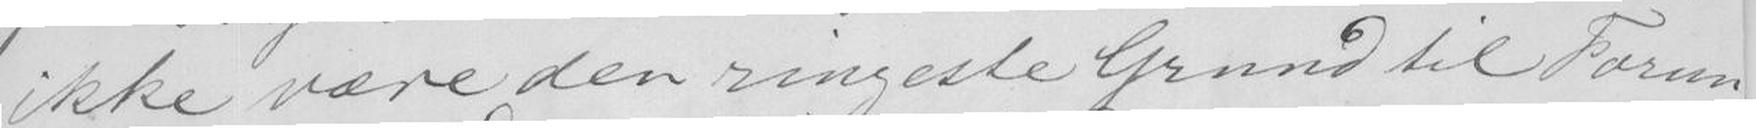ikke være den ringeste Grund til Forun-
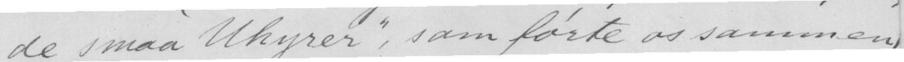de smaa Uhyrer, som førte os sammen),
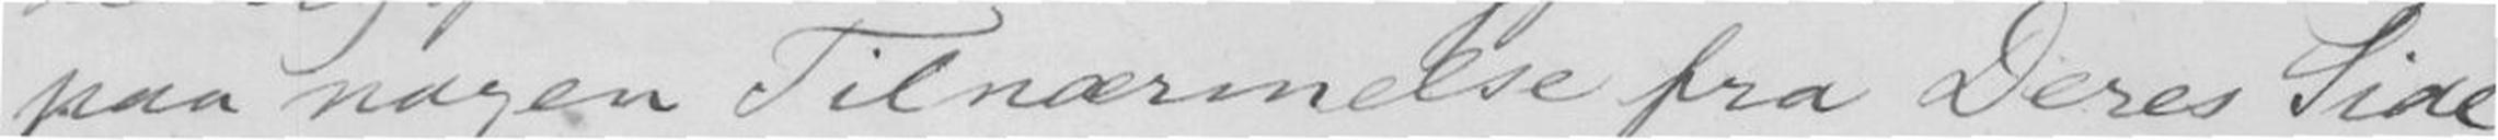paa nogen Tilnærmelse fra Deres Side,
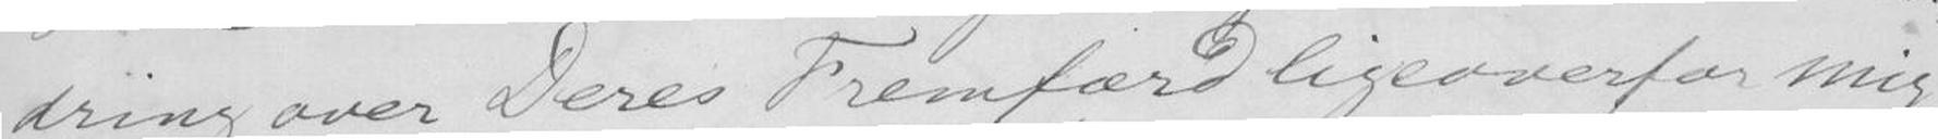dring over Deres Fremfærd ligeoverfor mig,
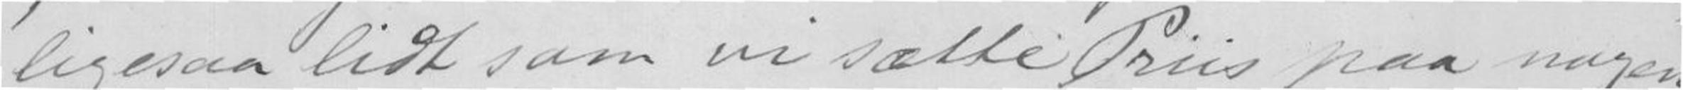ligesaa lidt som vi sætte Priis paa nogen
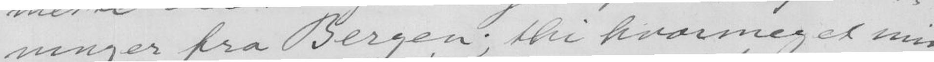ninger fra Bergen; thi hvormeget min
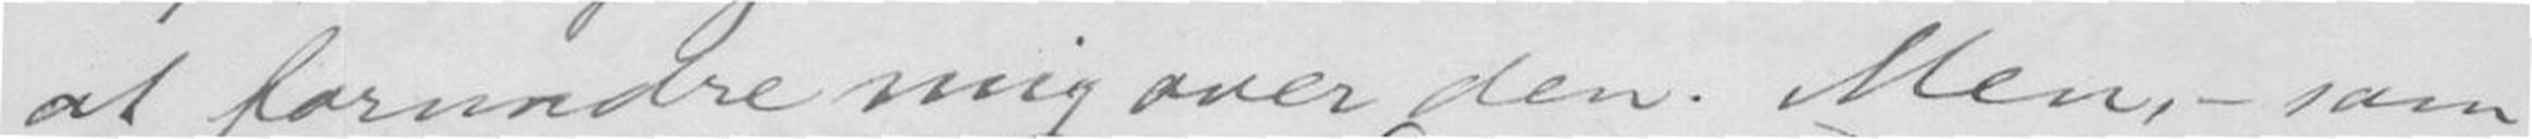at forundre mig over den. Men, - som
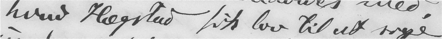hvad Hægstad fik lov til at sige
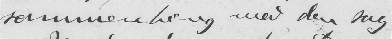sammenhæng med den sag.
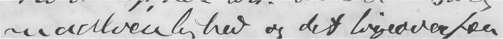maalvenlighed, og det ligeoverfor
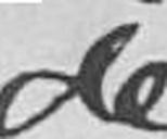de
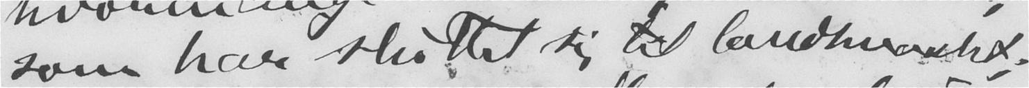som har sluttet sig til landsmaalet;
